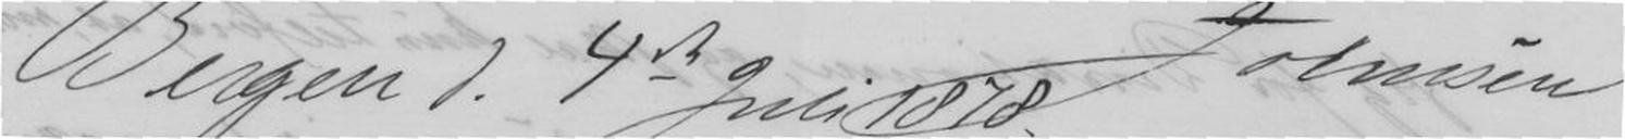Bergen d. 4de Juli 1878 Johnsen
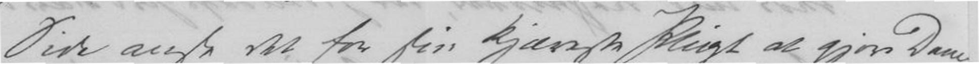Side anse det for sin kjæreste Pligt at gjøre Dem
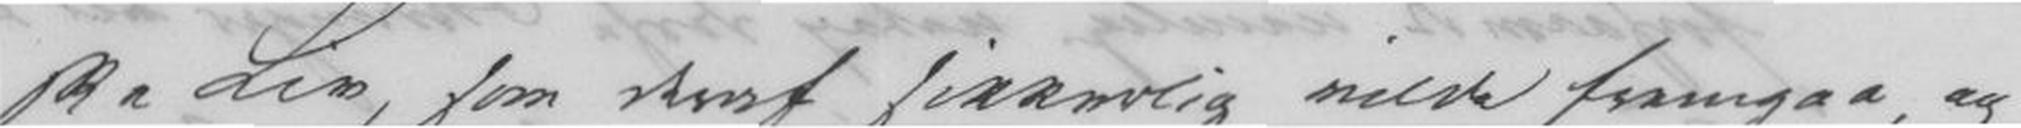ske Liv, som deraf sikkerlig vilde fremgaa, og
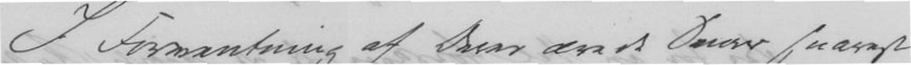I Forventning af Deres ærede Svar snarest
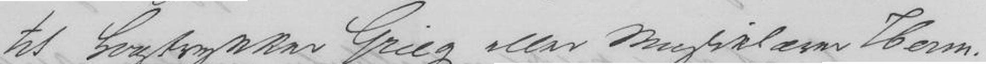til Bogtrykker Grieg eller Musiklærer Herm.
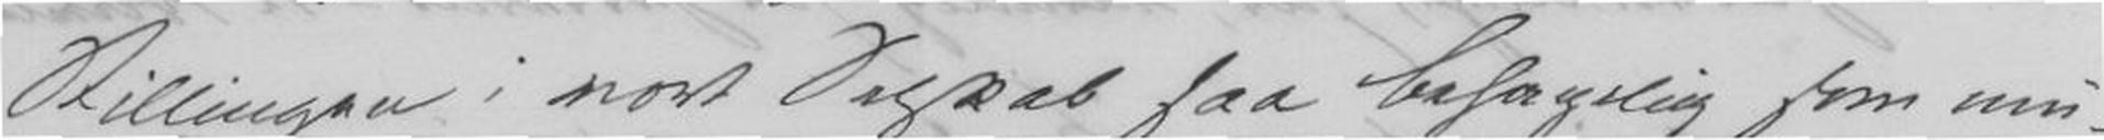Stillingen i vort Selskab saa behagelig som mu-
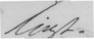ligt.
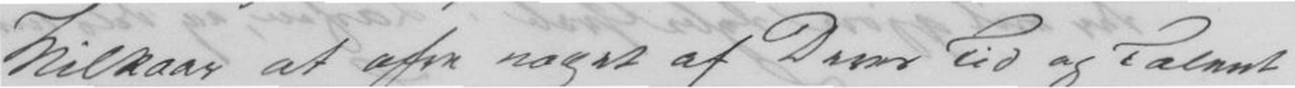Vilkaar at ofre noget af Deres Tid og Talent
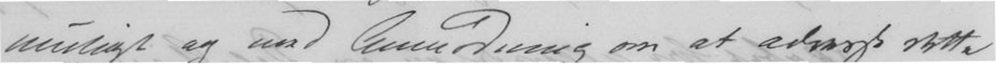muligt og med Anmodning om at adresse dette
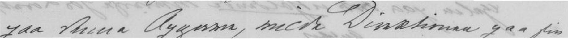paa denne Opgave, vilde Direktionen paa sin
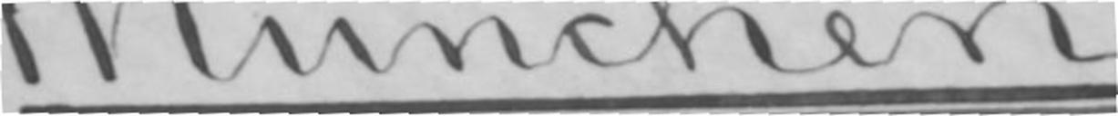München.
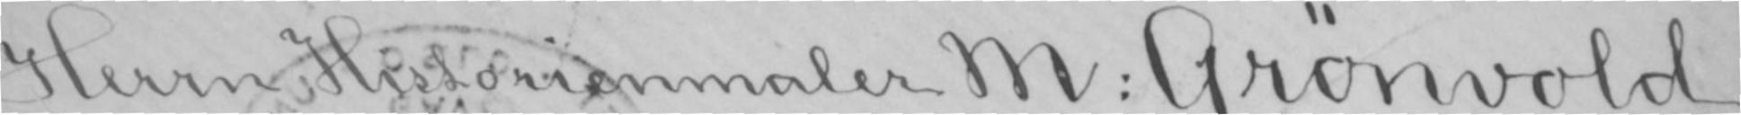Herrn Historienmaler M: Grønvold.
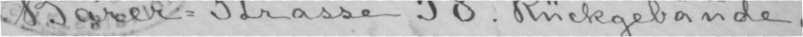Barer-Strasse 58. Rückgebäude.
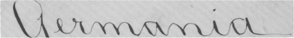Germania
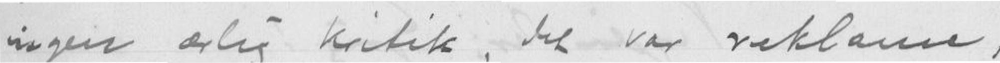ingen ærlig kritik, det var reklame,
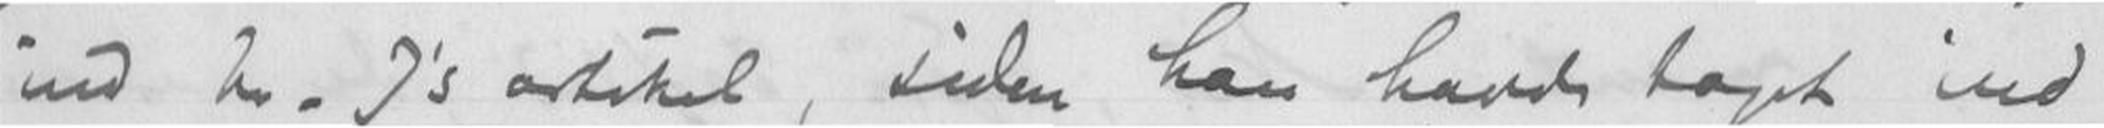ind hr. I's artikel, siden han havde taget ind
